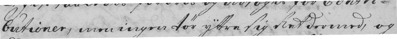butioner, men ingen tør yttre sig Ret dermed, og
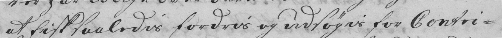at Fisk saaledis fordris og udsøgis for Contri-
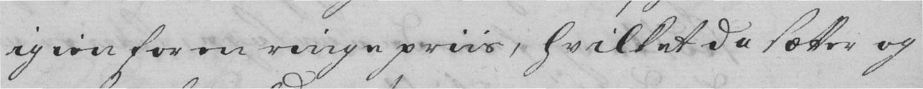igien for en ringe priis, hvilket de sætter og
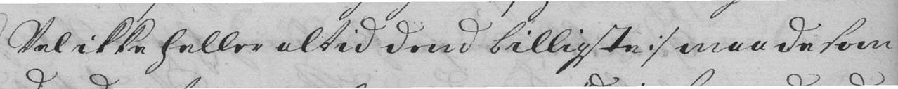Vel ikke heller altid dend billigste :/ maa de som
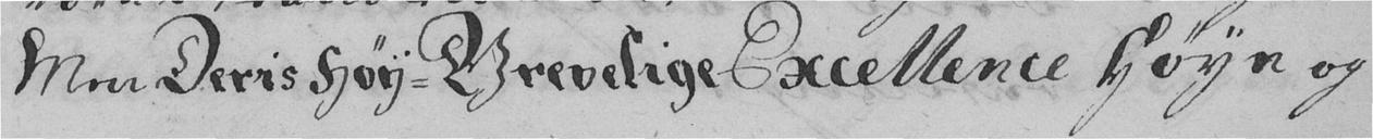Men Deris Høy-Grevelige Excellence Høye og
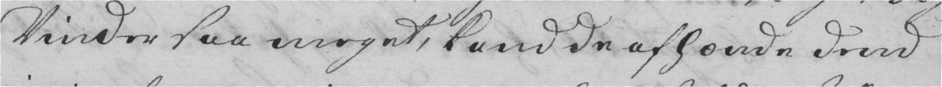Vinder saa meget, kand de afhænde dend
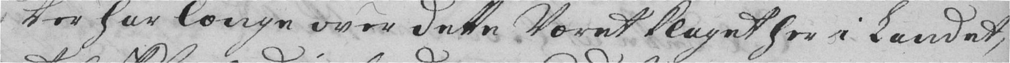Der har længe over dette Været klaget her i Landet,
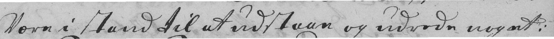være i stand til at udstaae og udrede noget.
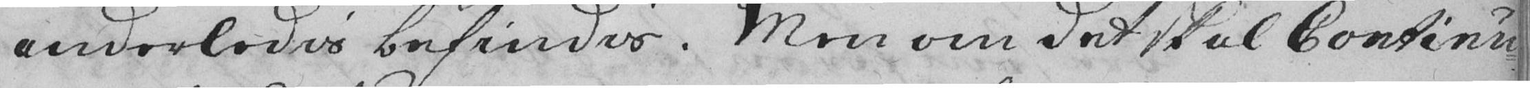anderledis befindis. Men om det skal Continu-
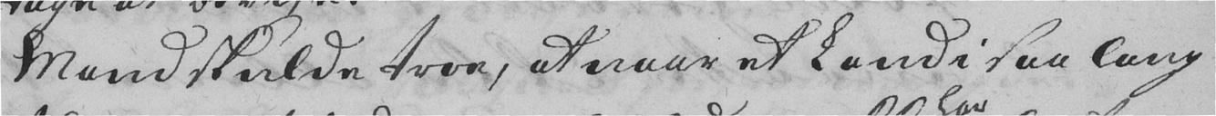Mand skulde troe, at naar et Land i saa lang
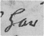har
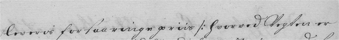leveris for saa ringe priis /: hvorved Vegten er
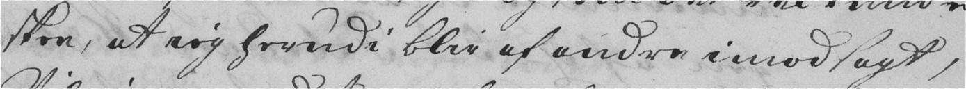skee, at ieg herudi blir af andre imodsagt,
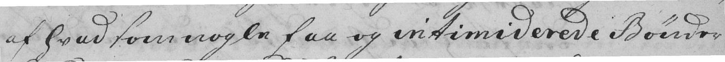af hvad som nogle faa og intimiderede Bønder
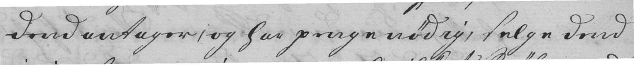dend antager, og har penge nødig, selge dend
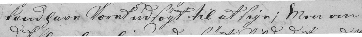kand have Været udsøgt til at sige; Men om
